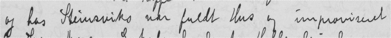og hos Steinsviks var fuldt Hus og improviseret
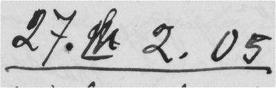27. 2.05
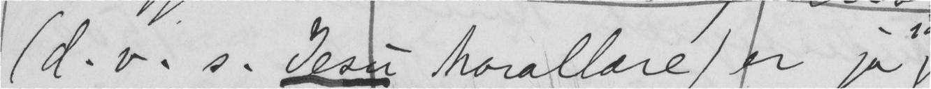(d. v. s. Jesu Morallære) er jo
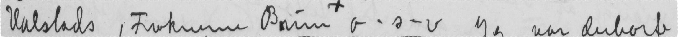Halstads, Frøknerne Brun o. s.v. Jeg var derborte
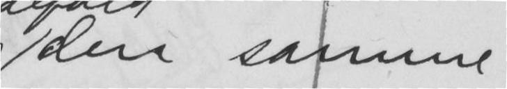den samme
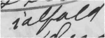ialfald
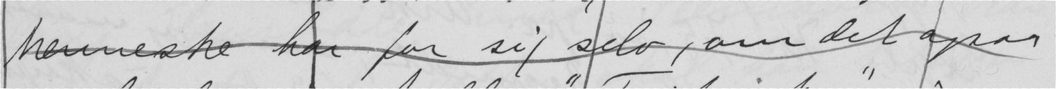Menneske har for sig selv, om det ogsaa
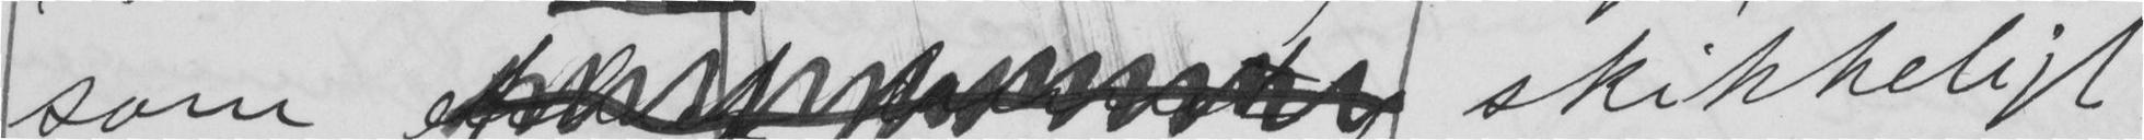som  skikkeligt
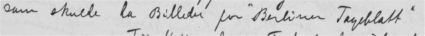som skulde ha Billeder for "Berliner Tageblatt"
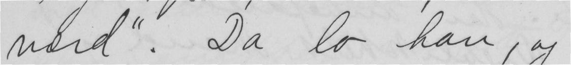værd". Da lo han, og saast
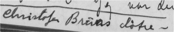Christofer Bruns døtre -
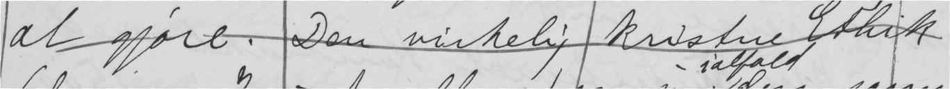at gjøre. Den virkelig kristne Ethik
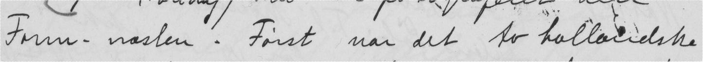Form. næsten. Først var det to hollandske
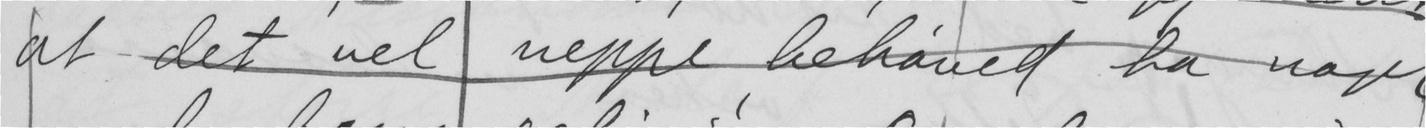at det vel neppe behøved ha noget
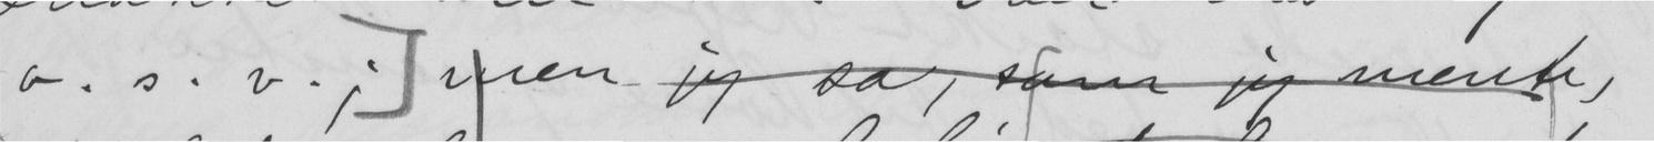o. s. v.; men jeg sa, som jeg mente,
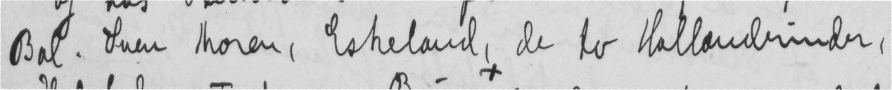Bol. Sven Moren, Eskeland, de to Hollanderinder,
